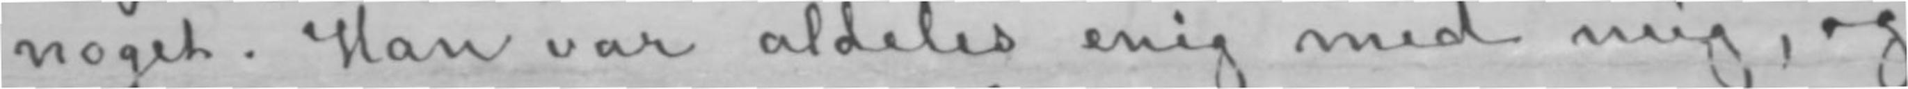noget. Han var aldeles enig med mig, og
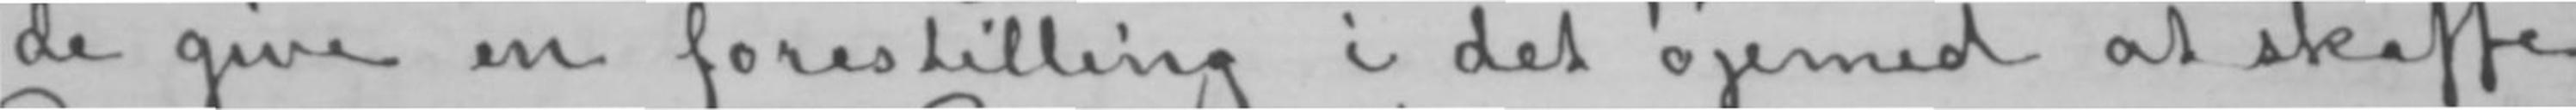de give en forestilling i det øjemed at skaffe
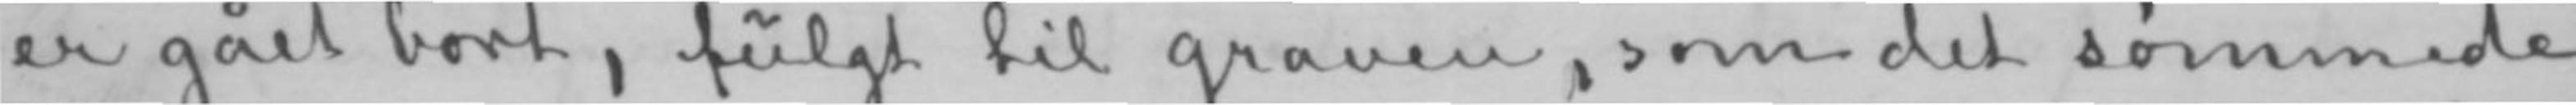er gået bort, fulgt til graven, som det sømmede
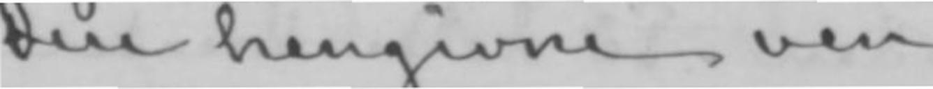Din hengivne ven
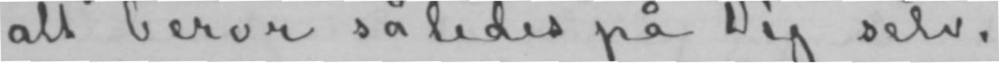alt beror således på Dig selv.
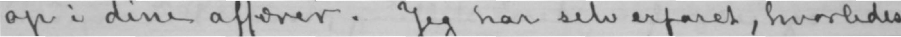op i dine affærer. Jeg har selv erfaret, hvorledes
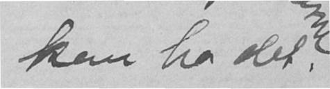kan ha det
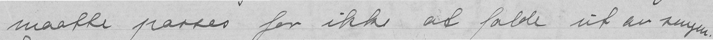maatte passes for ikke at folde ut av sengen.
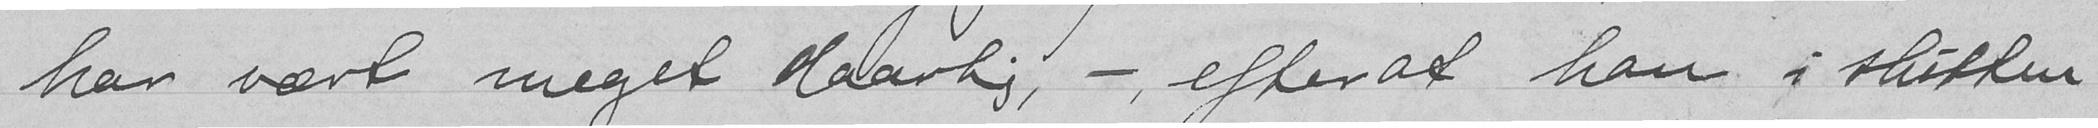har vært meget daarlig, -, efterat han i slutten
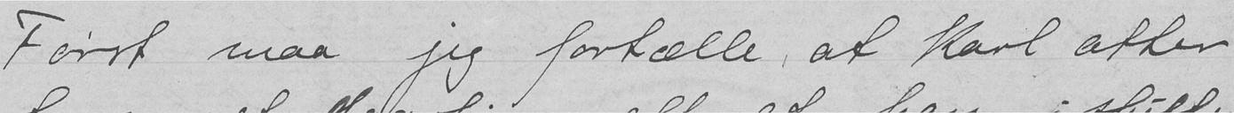Først maa jeg fortælle, at Karl atter
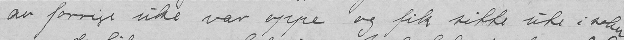ar forrige ude var oppe og fik sitte ute i solen
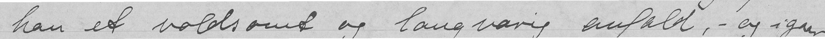han er voldsomt og langvarig anfald,- og igaar
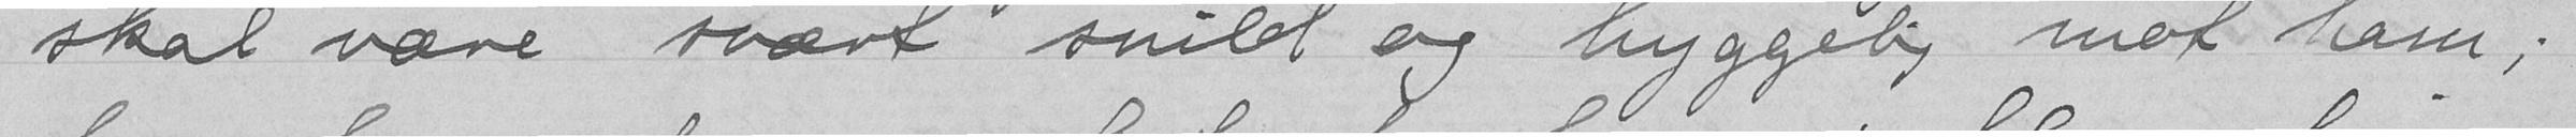skal være svart snild og hyggelig mot ham;
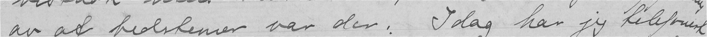av at bedstemer var der. Idag har jeg telefonert
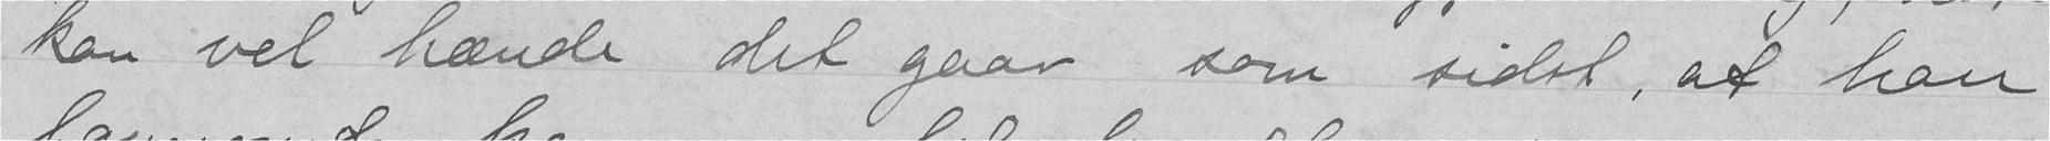kan vel hænde det gaar som sidst, at han
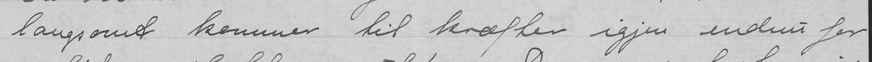langsomt kommer til kræfter igjen endnu for
